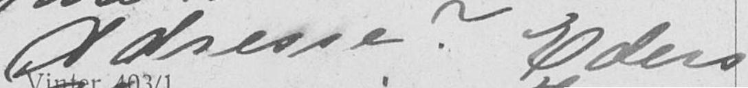Adresse? Eders
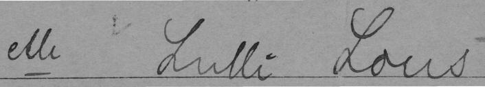elle Lulli Lous
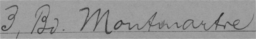3, Bd. Montmartre
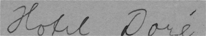Hotel Doré
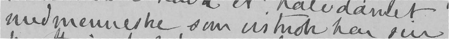medmenneske som vistnok har sin
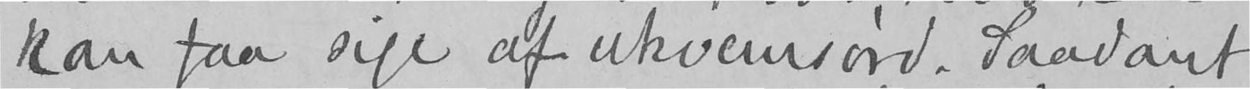kan faa sige af ukvemsord. Saadant
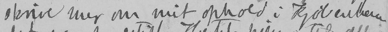skrive mer om mit ophold i Kjøbenhavn
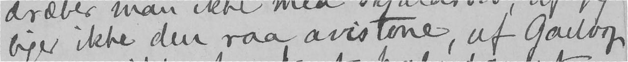liger ikke den raa avistone, uf Garborg
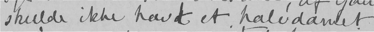skulde ikke havt et halvdannet
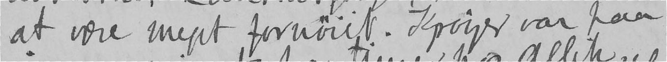at være meget fornøiet. Krøyer var paa
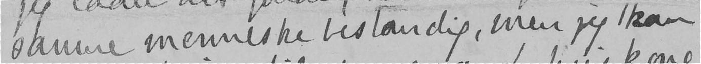samme menneske bestandig, men jeg kan
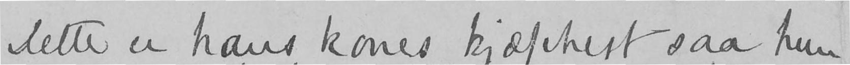Dette er hans kones kjæphest, saa hun
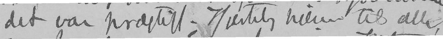det var prægtigt. Hjertelig hilsen til alle,
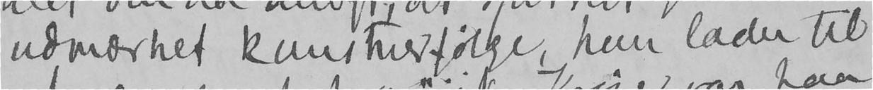udmærket kunstnerfølge, hun lader til
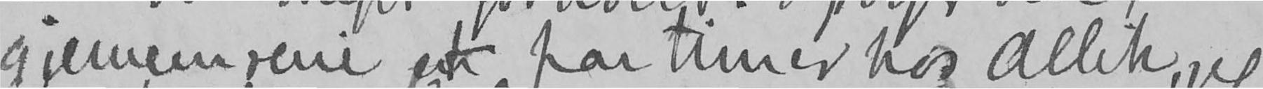gjennemreise et par timer hos Allik, jeg
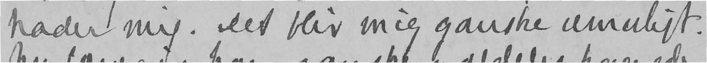hader mig. Det blir mig ganske umuligt.
